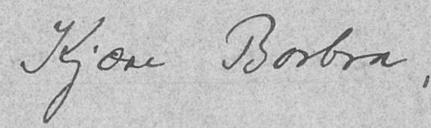Kjære Barbra,
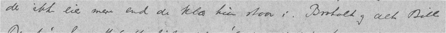der ikke eier mere end de klær hun staar i.  Broholt og alt Bilde
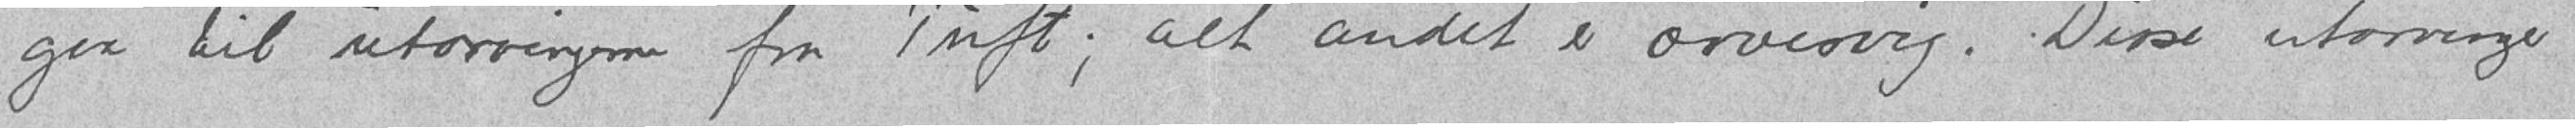gaa til utarvingerne fra Tuft; alt andet er arvesvig. Disse utarvinger
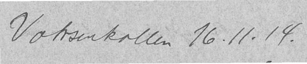Voksenkollen 16.11.14.
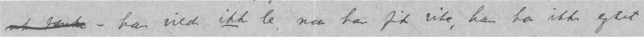at tænke – han vilde ikke le, naar han fik vite, han har ikke egtet
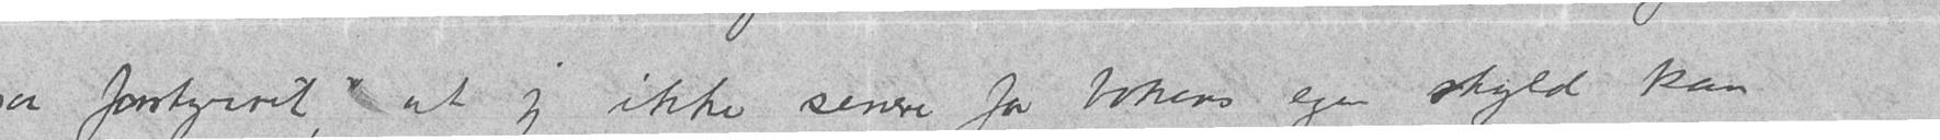i maaneskin «den røde mund synes endda rødere» – saa blir ialfald
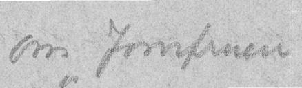om "Jomfruen"
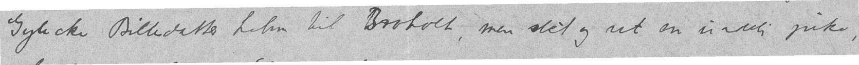Gylicke Bildedatter Lehm til Broholt, men slet og ret en uadelig pike,
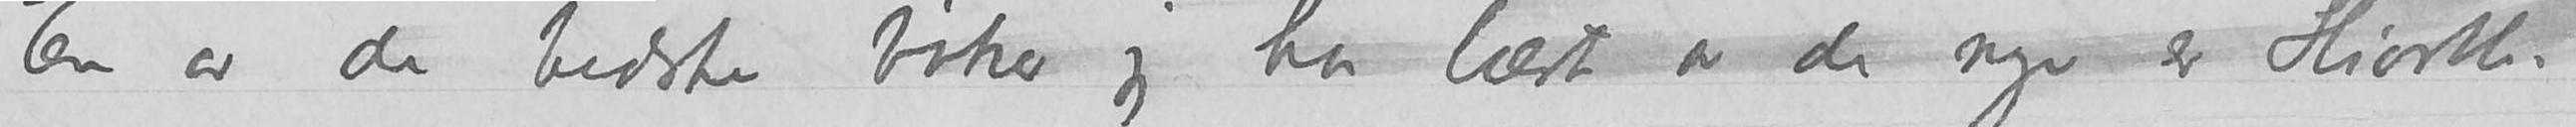En av de bedste bøker jeg har læst av de nye er Hiorth-
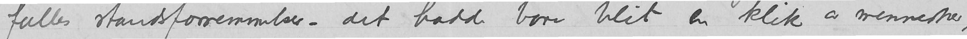fælles standsfornemmelser – det hadde bare blit en klik av mennesker,
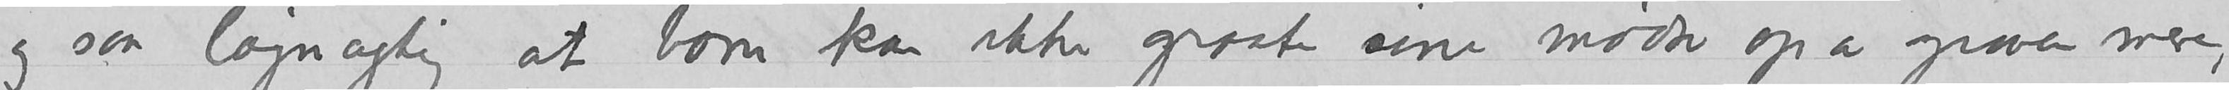og saa løgnagtig at barn kan ikke graate sine mødre op av graven mere,
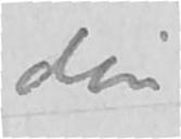din
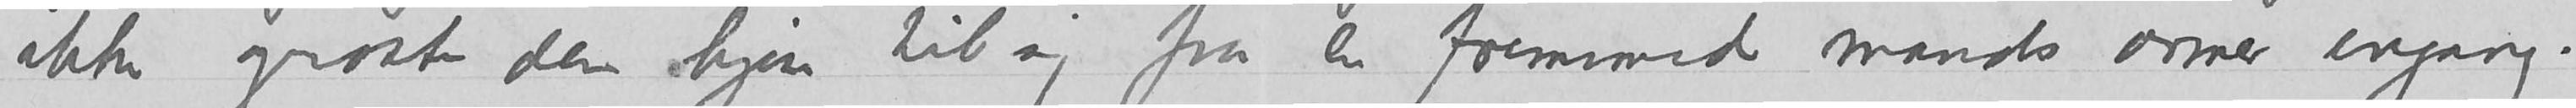ikke graate dem hjem til sig fra en fremmed mands armer engang.
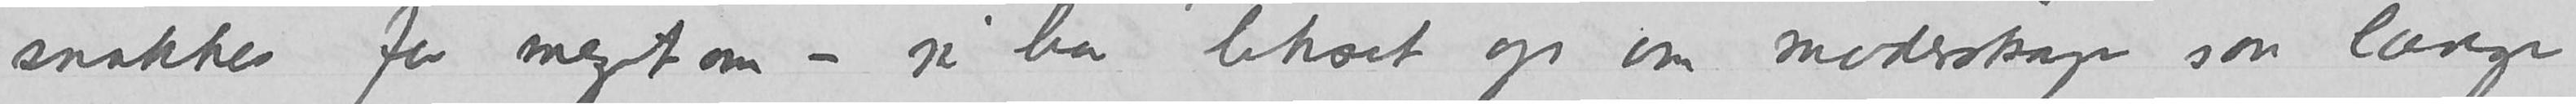snakkes for meget om – jeg har lekset op om moderskap saa længe
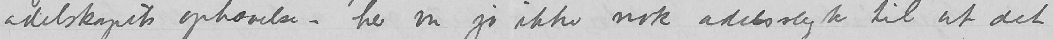adelskapets ophævelse – her var jo ikke nok adelsslegter til at det
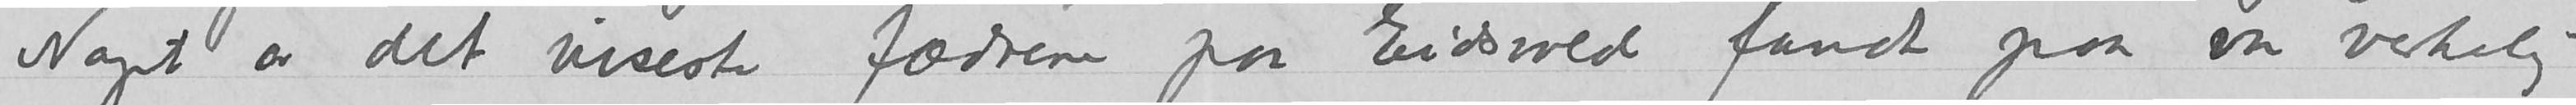Noget av det viseste fædrene på Eidsvold fandt paa var virkelig
